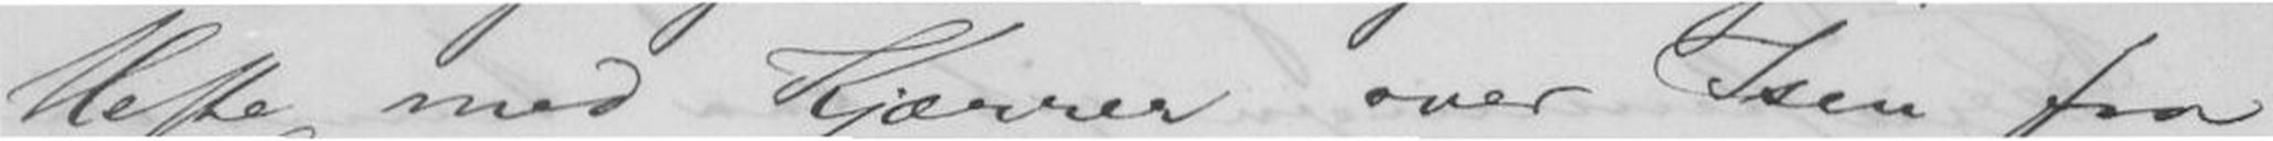Heste med Kjærrer over Isen fra
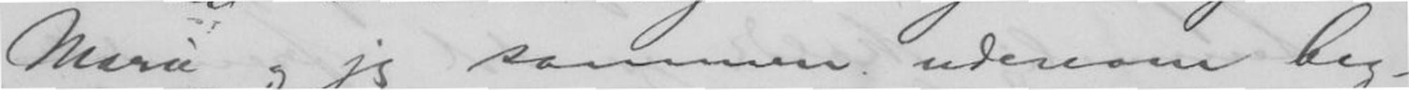Marie og jeg sammen udenom beg-
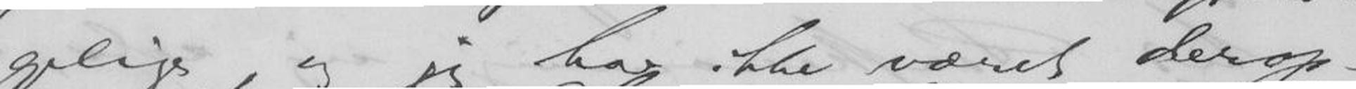gelige, og jeg har ikke været derop-
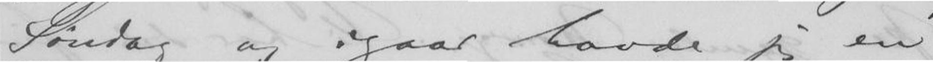Søndag og igaar havde jeg en
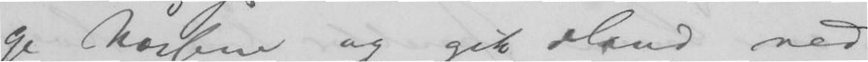ge Næssene og gik iland ved
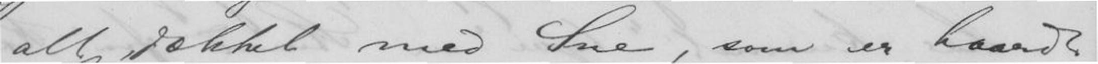alt dækket med Sne, som er haardt
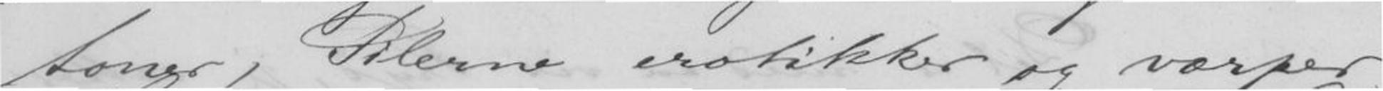toner, Pilerne erotikker og værper,
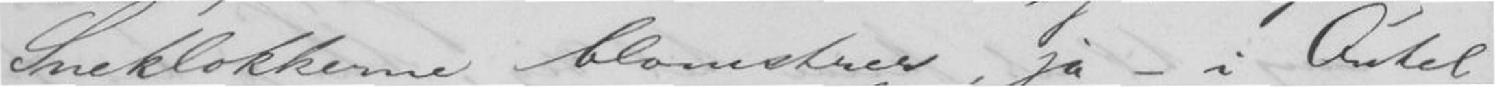Sneklokkerne blomstrer, ja - i Onkel
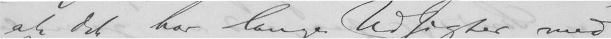at det har lange Udsigter med
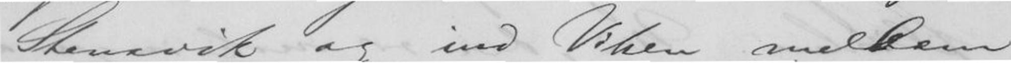Stensvik og ind Viken mellem
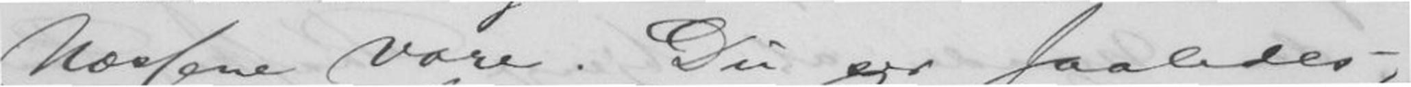Næssene vore. Du ser saaledes,
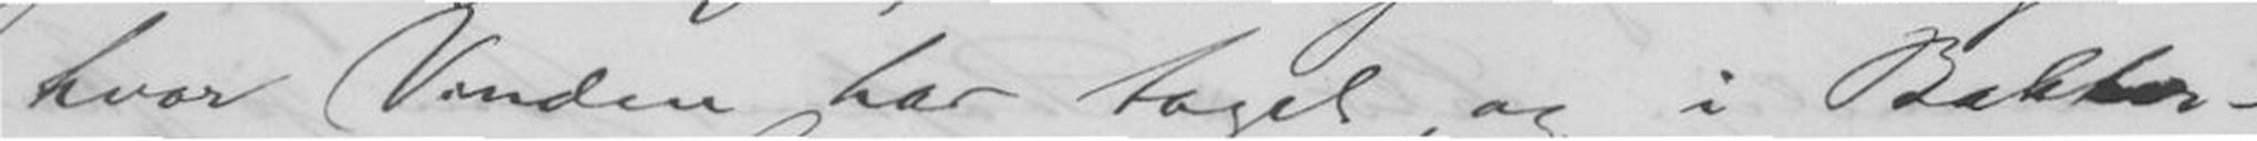hvor Vinden har taget, og i Bakker-
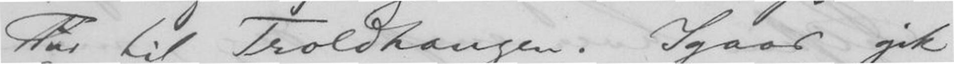Tur til Troldhaugen. Igaar gik
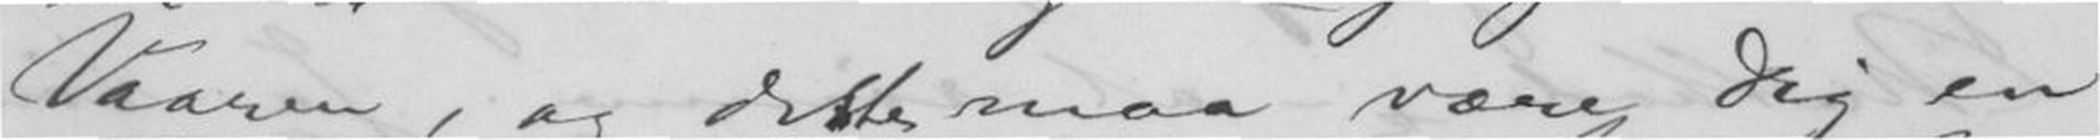Vaaren, og dette maa være Dig en
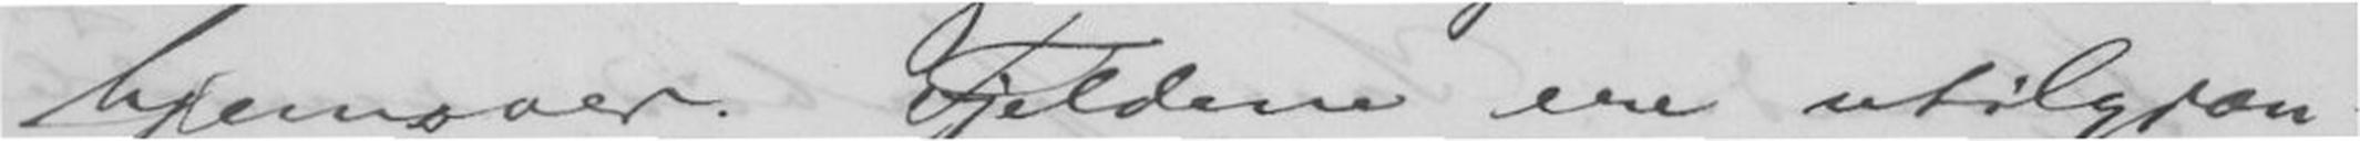hjemover. Fjeldene ere utilgjæn-
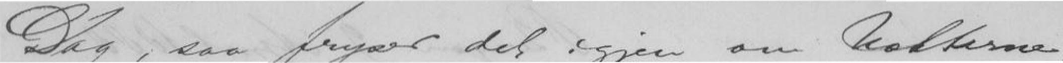Dag, saa fryser det igjen om Nætterne,
